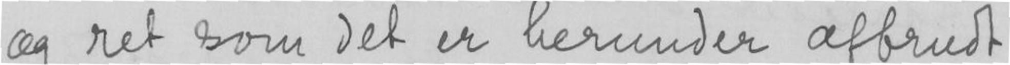og ret som det er herunder afbrudt
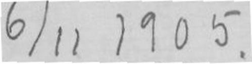6/11 1905.
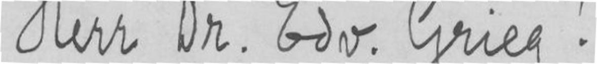Herr Dr. Edv. Grieg!
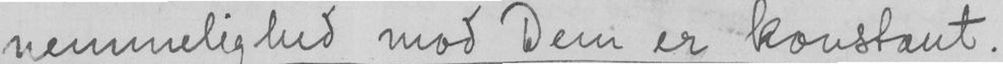nemmelighed mod Dem er konstant.
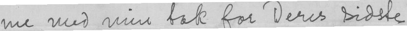me med mine tak for Deres sidste
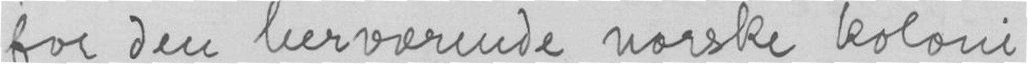for den herværende norske koloni
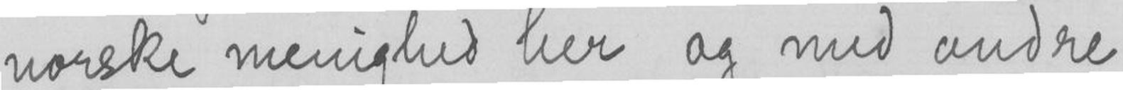norske menighed her og med andre
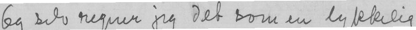Og selv regner jeg det som en lykkelig
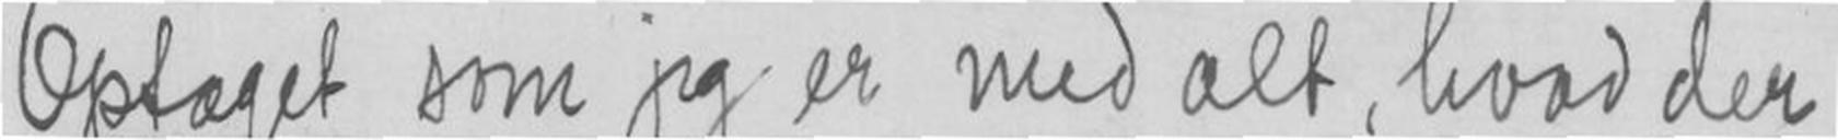Optaget som jeg med alt, hvad der
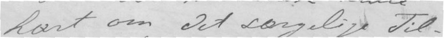hørt om det sørgelige Til-
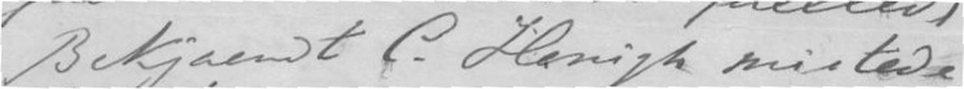Bekjaendt C. Henigh mistede
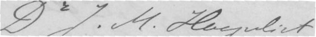D J.M.Hoogenlict
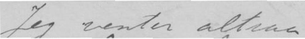Jeg venter altsaa
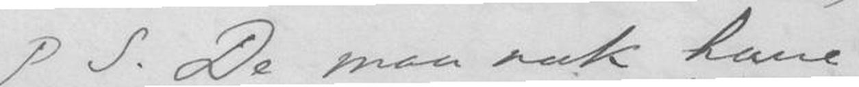P.S. De maa nok have
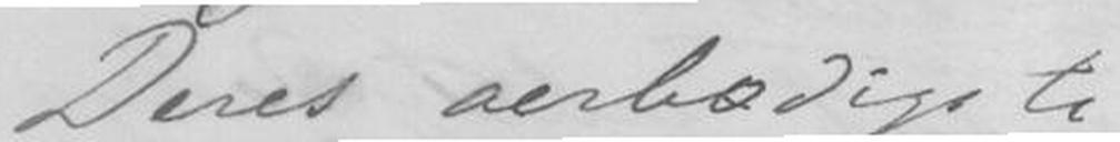Deres aerbødigste
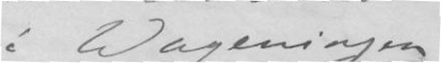i Wageningen
